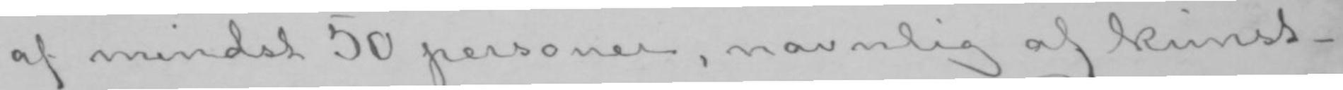af mindst 50 personer, navnlig af kunst-
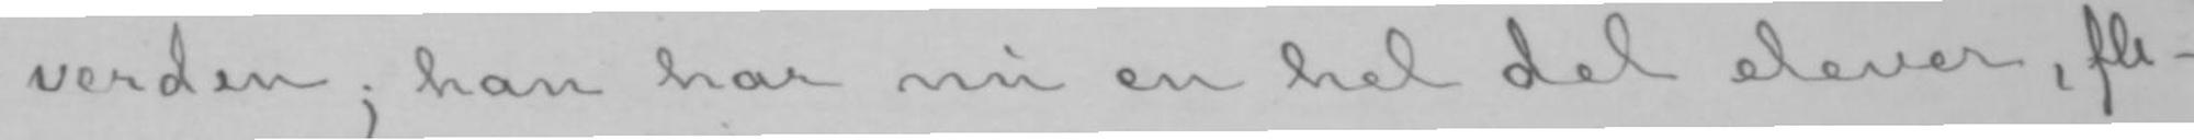verden; han har nu en hel del elever, fle-
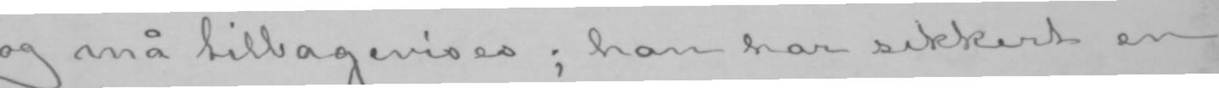og må tilbagevises; han har sikkert en
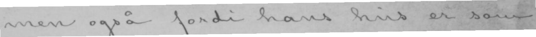men også fordi hans hus er som
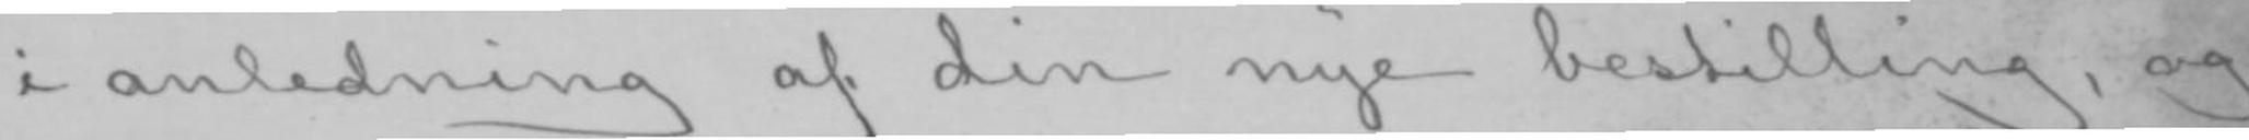i anledning af din nye bestilling, og
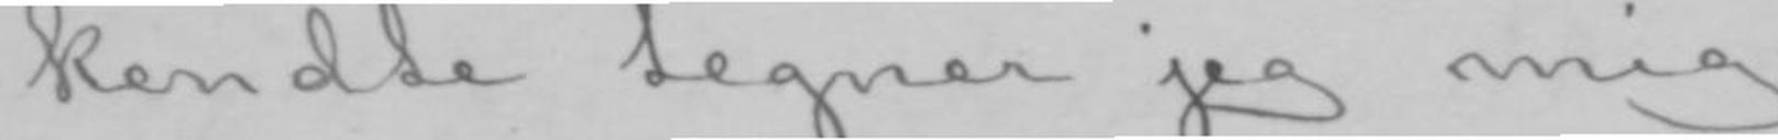kendte tegner jeg mig
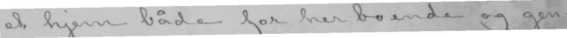et hjem både for herboende og gen-
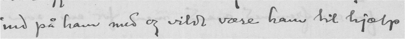ind på ham med og vilde være ham til hjælp
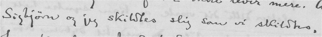Sigbjørn og jeg skildtes slig som vi skildtes,
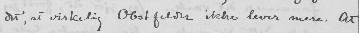det, at virkelig Obstfelder ikke lever mere. At
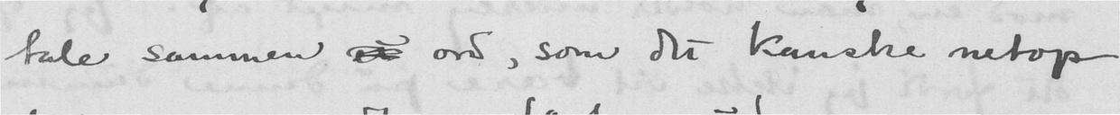tale sammen et ord, som det kanske netop
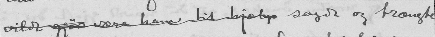vilde gjøre være ham til hjælp sagde og trængte
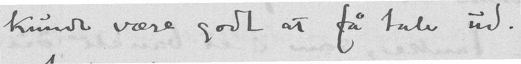kunde være godt at få tale ud.
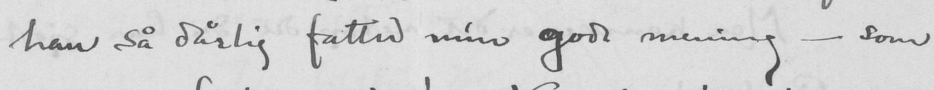han så dårlig fatted min gode mening - som
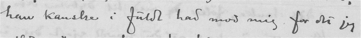han kanske i fuldt had mod mig for det jeg
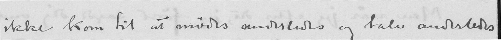ikke kom til at mødes anderledes og tale anderledes,
